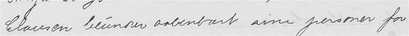Clausen beundrer aabenbart sine personer for
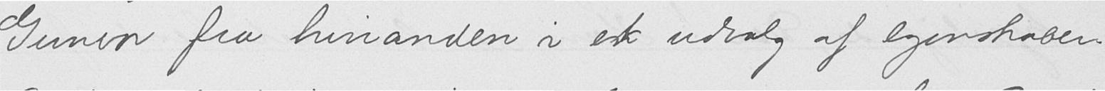Gunvor fra hinanden i et udvalg af egenskaber.
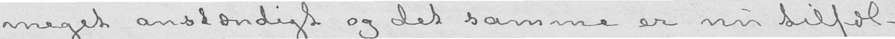meget anstændigt og det samme er nu tilfæl-
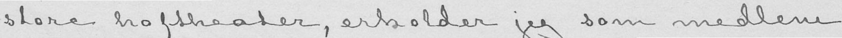store hoftheater, erholder jeg som medlem
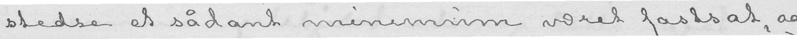stedse et sådant minimum været fastsat, og
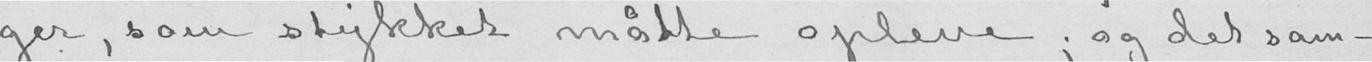ger, som stykket måtte opleve; og det sam-
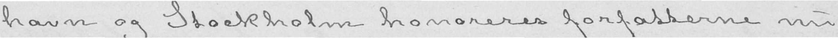havn og Stockholm honoreres forfatterne nu
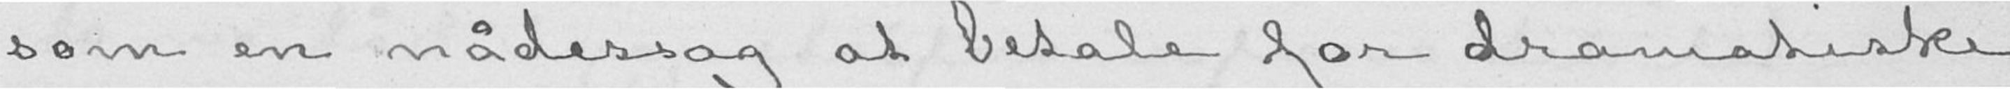som en nådessag at betale for dramatiske
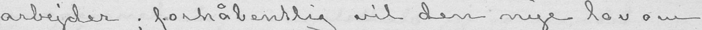arbejder; forhåbentlig vil den nye lov om
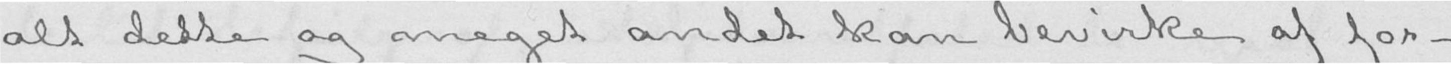alt dette og meget andet kan bevirke at for-
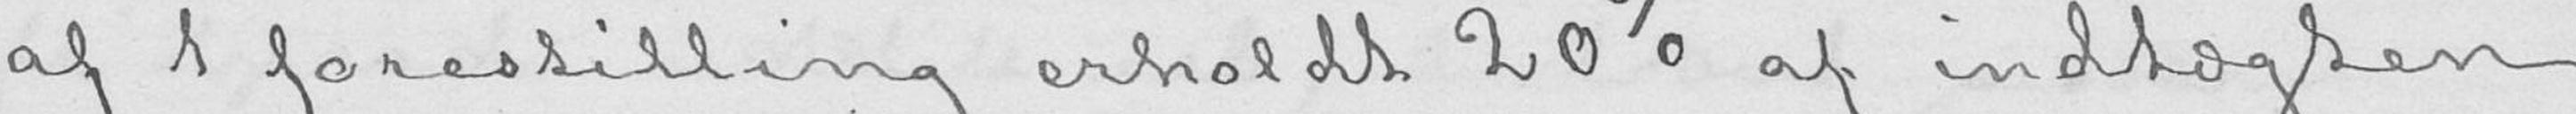af 1 forestilling erholdt 20 % af indtægten
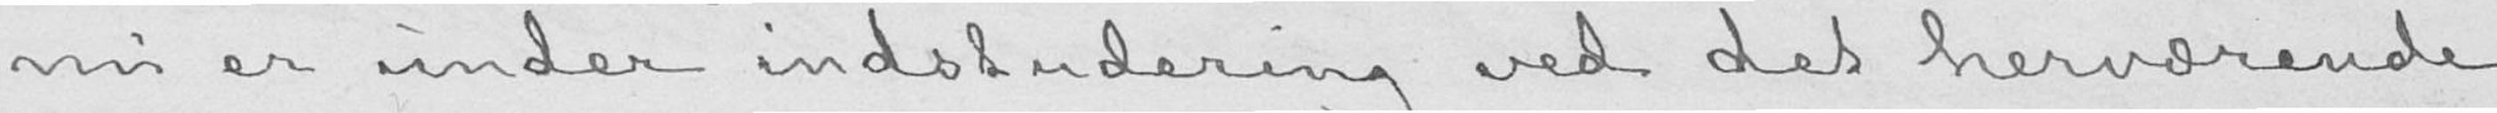nu er under indstudering ved det herværende
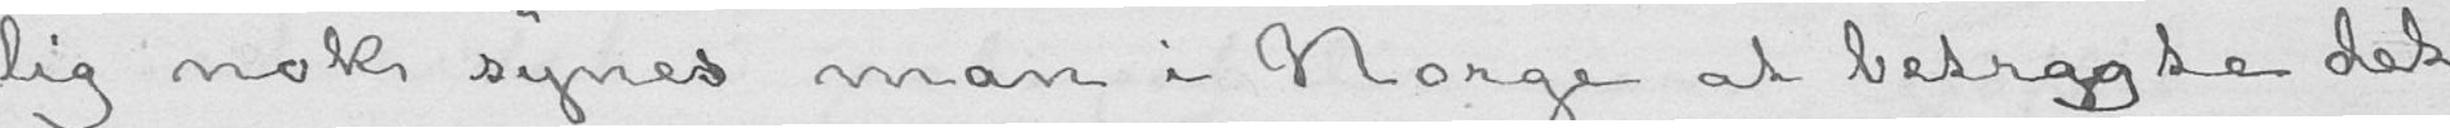lig nok synes man i Norge at betrgagte det
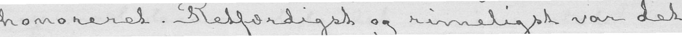honoreret. Retfærdigst og rimeligst var det
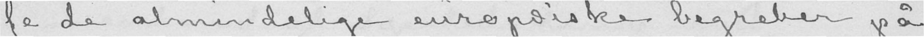fe de almindelige europæiske begreber på
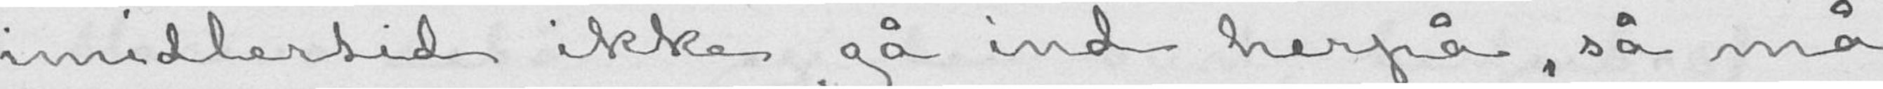imidlertid ikke gå ind herpå, så må
